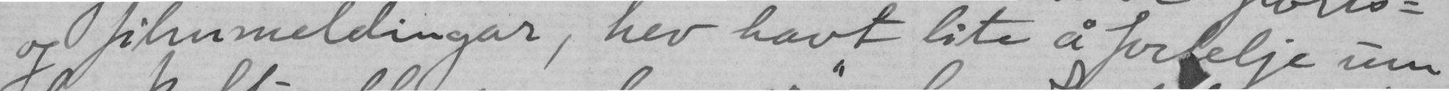og filmmeldingar, hev havt lite å fortelje um
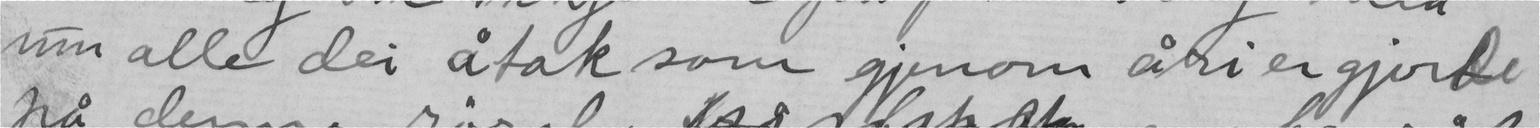um alle dei åtak som gjenom åri er gjort
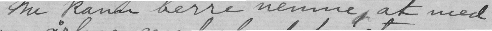Me kann berre nemne, at med
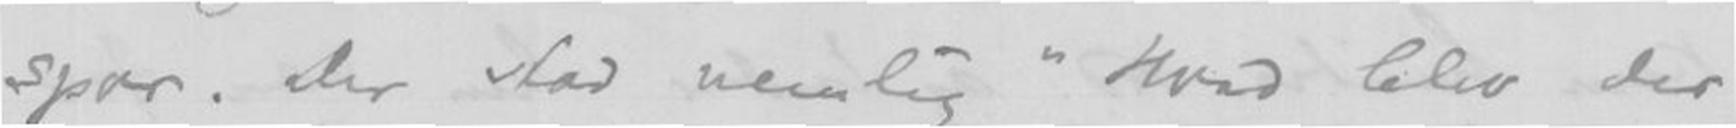spor. Der stod nemlig "hvad blev der
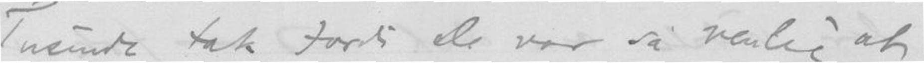Tusinde tak fordi De var så venlig at
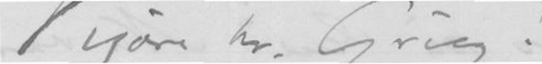Kjære hr. Grieg!
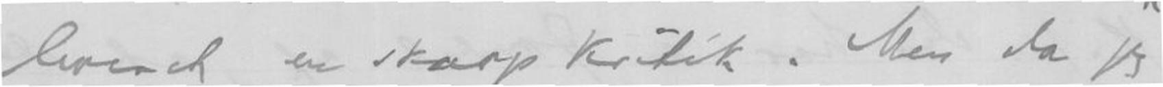leveret en skarp kritik. Men da jeg
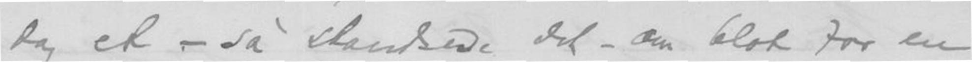dag et - så stansede det - om blot for en
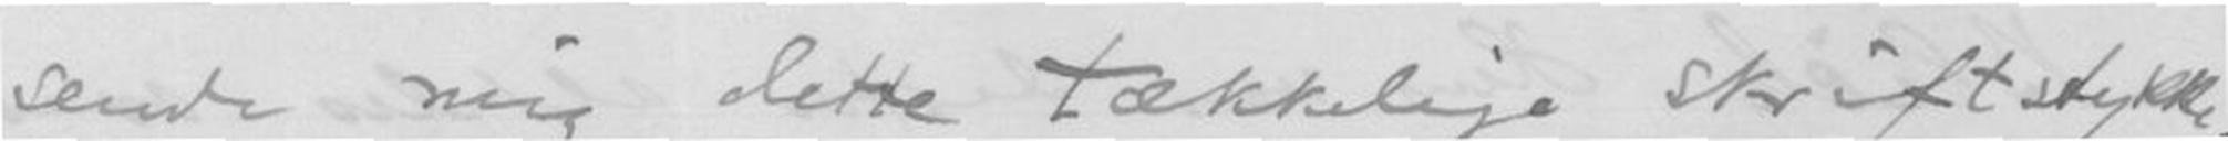sende mig dette tækkelige skriftstykke.
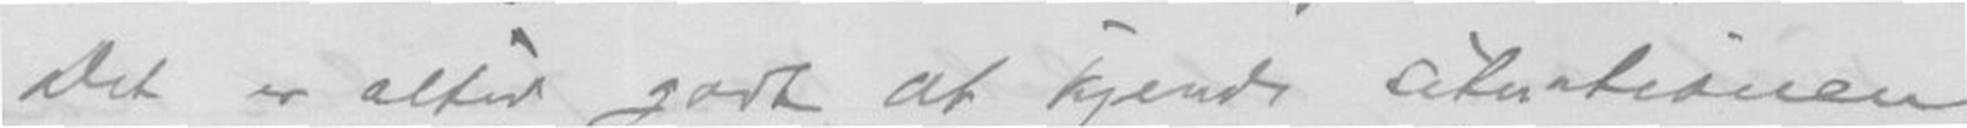Det er altid godt at kjende situationen
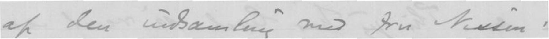af den indsamling med fru Nissen i
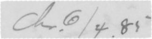Chr 6/4.85
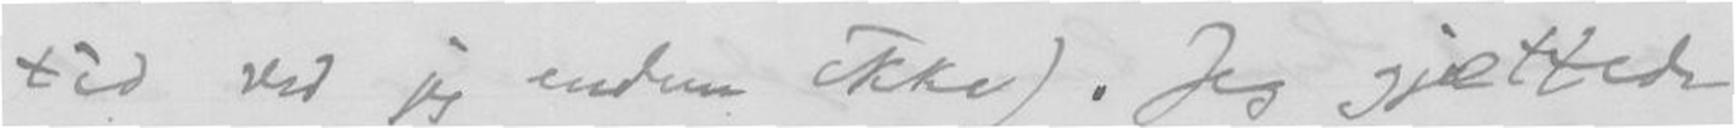tid ved jeg endnu ikke). Jeg gjættede
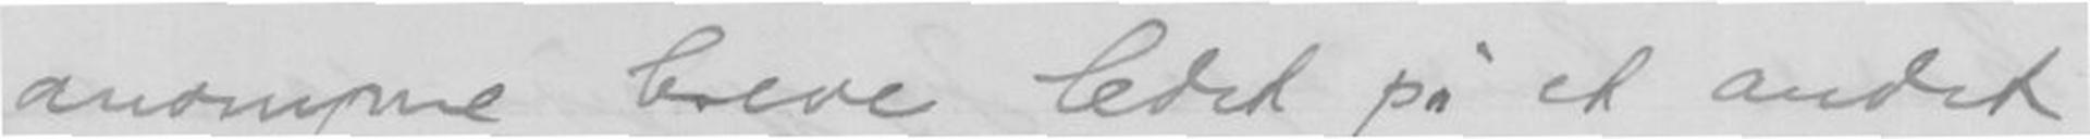anonyme breve ledet på et andet
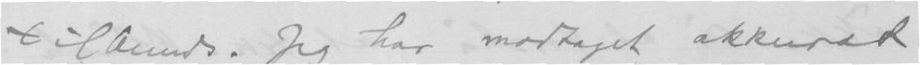tilbunds. Jeg har modtaget akkurat
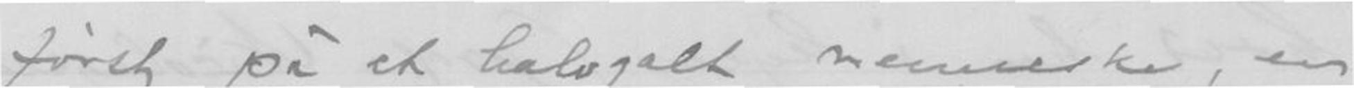først på et halvgalt menneske, en
